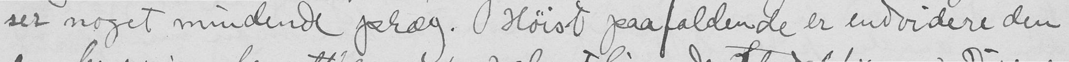ser noget mindende præg. Høist paafaldende er endvidere den
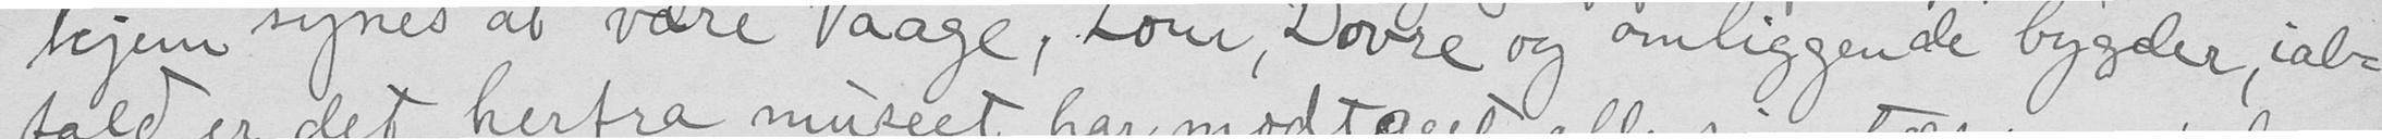hjem synes at være Vaage, Lom Dovre og omliggende bygder, ial-
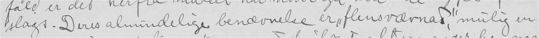slags. Deres almindelige benævnelse er "flensvævnad" mulig en
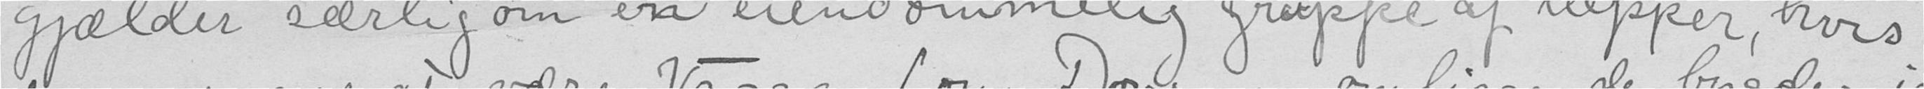gjælder særlig om en eiendommelig gruppe af tæpper, hvis
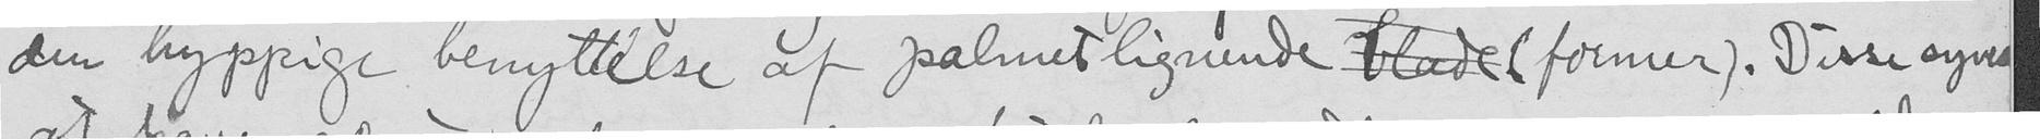den hyppige benyttelse af palmetlignende  (former). Disse synes
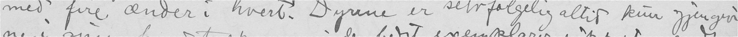med fire ænder i hvert. Dyrne er selvfølgelig altid kun gjengiv-
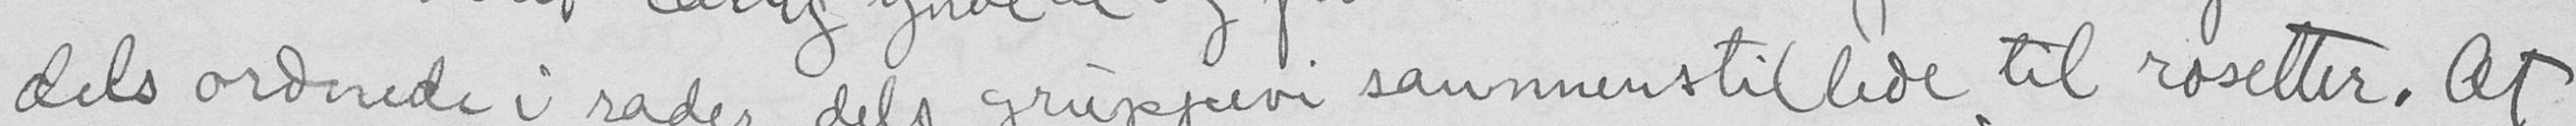dels ordnede i rader, dels grupperi sammenstillede til rosetter. At
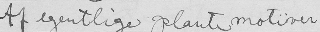Af egentlige plantemotiver
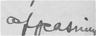afpasning
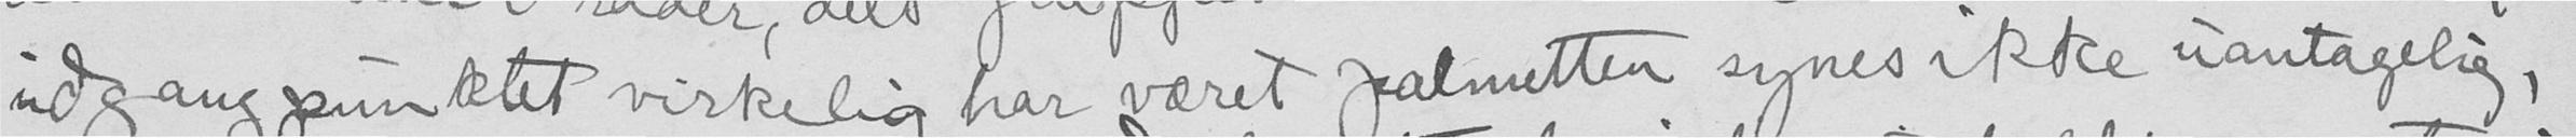udgangpunktet virkelig har været palmetten synes ikke uantagelig,
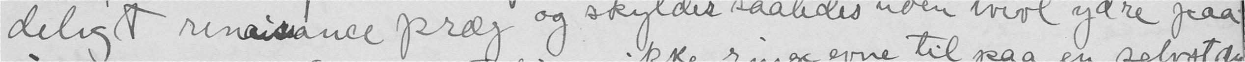deligt renaisance præg og skylder saaldes uden tvivl ydre paa-
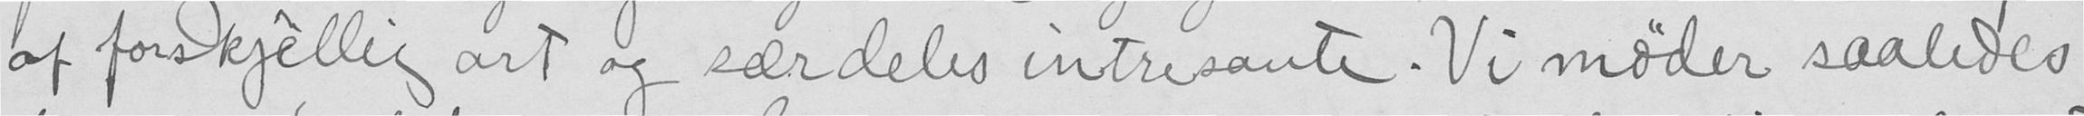af forskjellig art og særdeles intresante. Vi møder saaledes
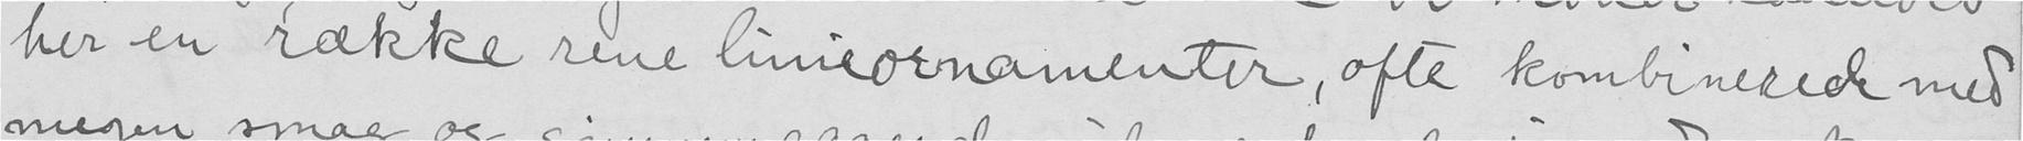her en række rene lineornamenter, ofte kombinerede med
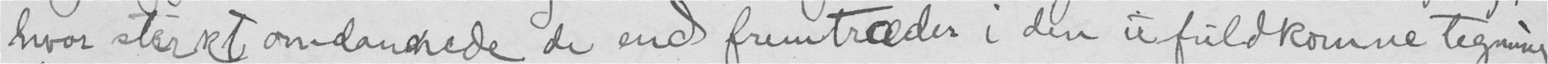hvor sterkt omdannede de end fremtræder i den ufuldkomne tegning
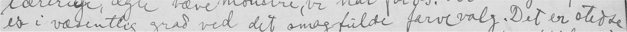es i væsentlig grad ved det smagfulde farvevalg. Det er stedse
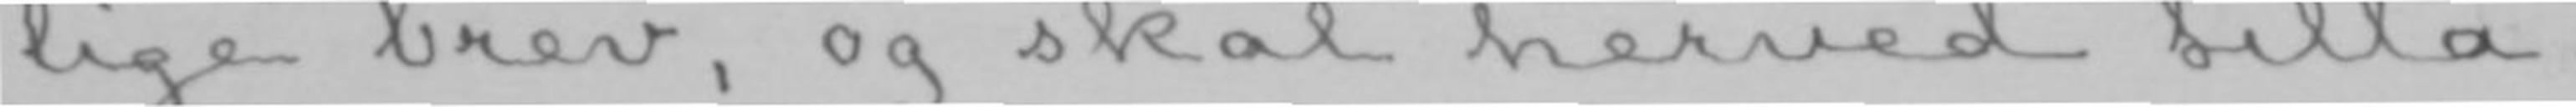lige brev, og skal herved tilla-
